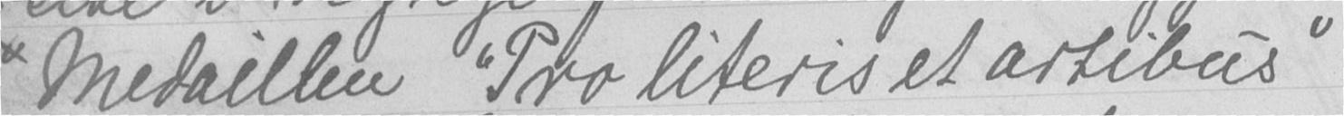Medaillen "Pro literis et artibus"
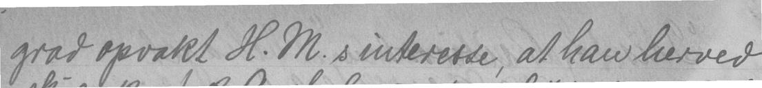grad opvakt H. M. s interesse, at han herved
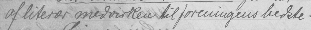af literær medvirken til foreningens bedste.
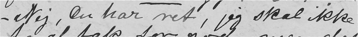- Nej, Du har ret, jeg skal ikke
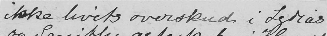ikke livets overskud i Lydias
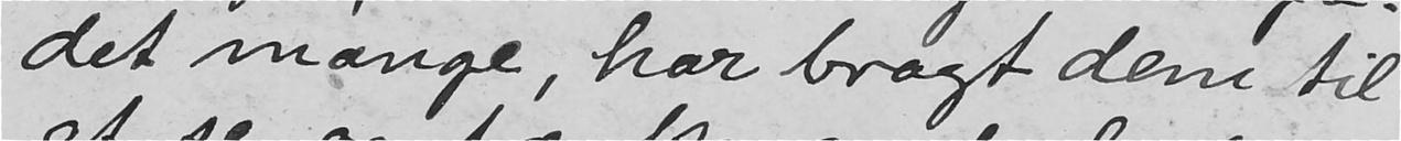det mange, har bragt dem til
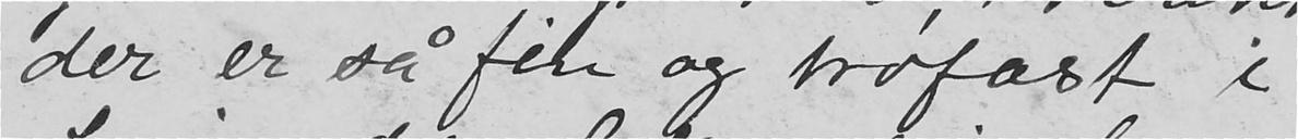der er du så fin og trofast i
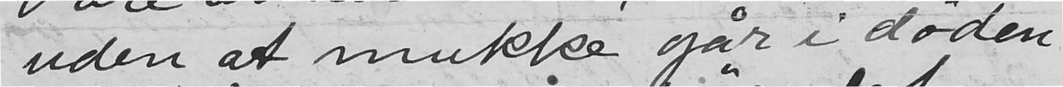uden at mukke går i døden
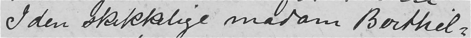I den skikkelige madam Berthel-
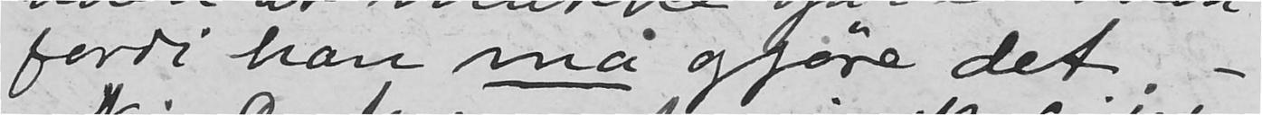fordi han må gjøre det. -
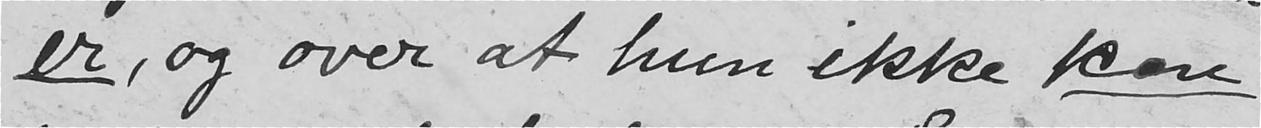er, og over at hun ikke kan
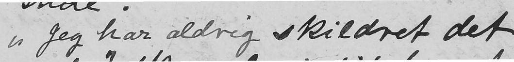"jeg har aldrig skildret det
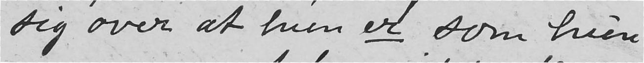sig over at hun er som hun
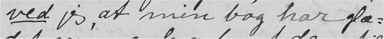ved jeg, at min bog har glæ-
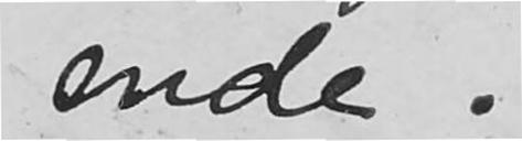ende.
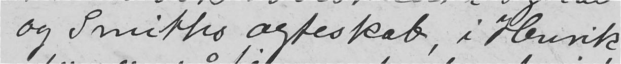og Smiths ægteskab i Henrik
